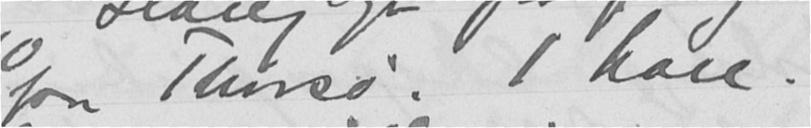paa Thorsø. 1 hare.
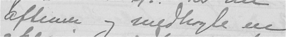aftenen og medbragte en
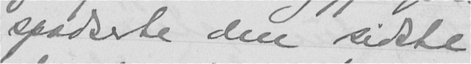spadserte den sidste
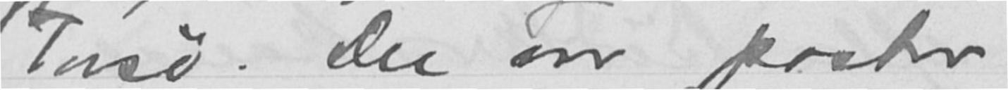Torsø. Der var pastor
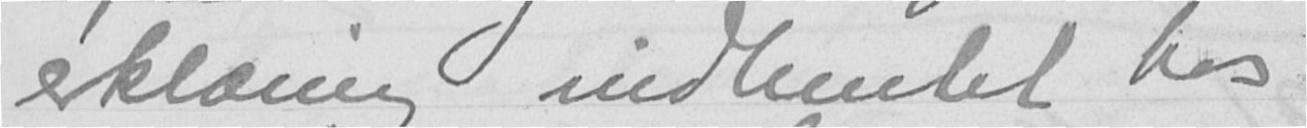erklæring indhentet hos
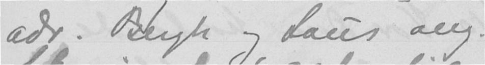adv. Bergh og Laus ang.
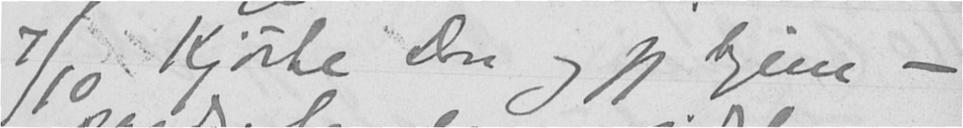7/10 Kjørte Don og jeg hjem -
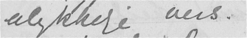ulykkelige vers.
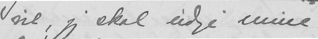vil, jeg skal udgi mine
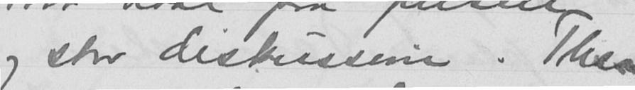og stor diskussion. Thea
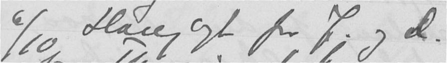6/10 Harejagt for J. og D.
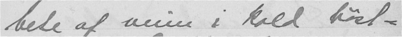bete af veien i kold høst-
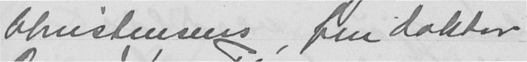Christensens, fru doktor
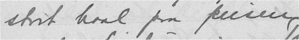stort baal paa peisen
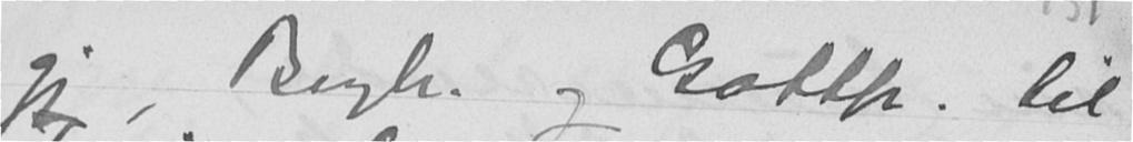jeg, Borgh. og Gottfr. til
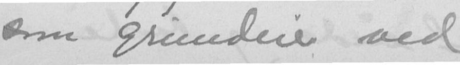som grundeier ved
